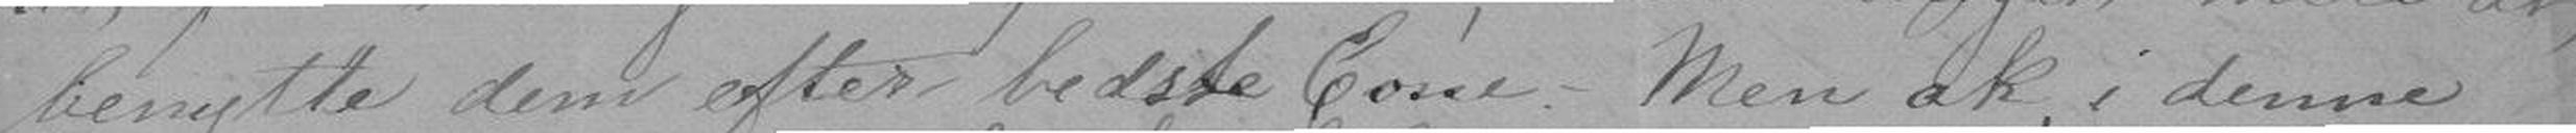benytte dem efter bedste Evne. - Men ak, i denne
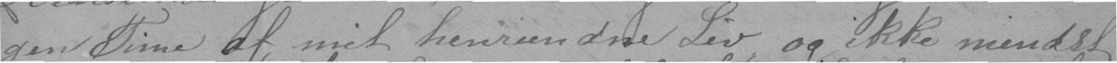gen Time af mit henrundne Liv, og ikke mindst
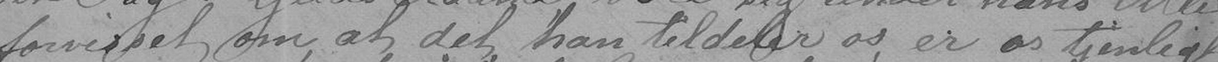forvisset om, at det han tildeler os, er os tjenligt
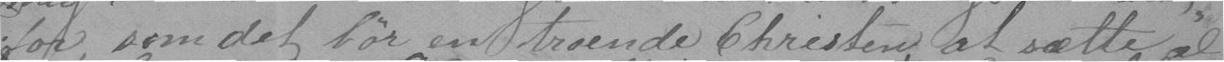for, som det bør en troende Christen at sætte al
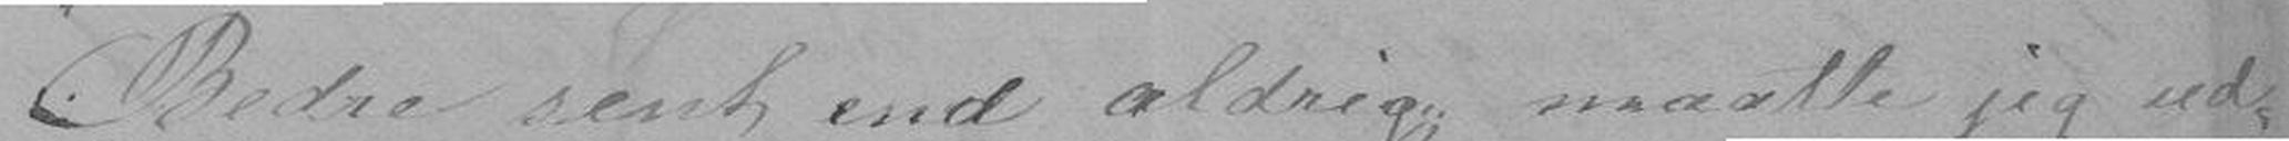Bedre sent end aldrig; maatte jeg ud-
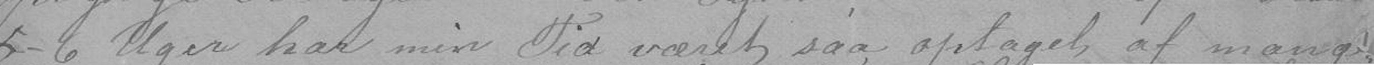5-6 Uger har min Tid været saa optaget af mange
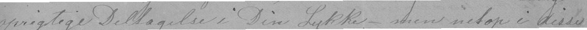oprigtige Deltagelse i Din Lykke, - men netop i disse
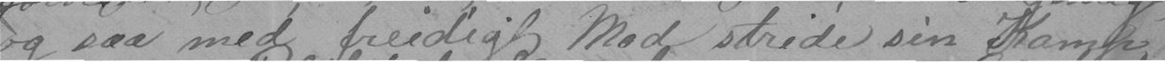og saa med freidigt Mod stride sin Kamp
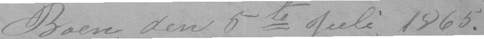Boen, den 5te Juli 1865.
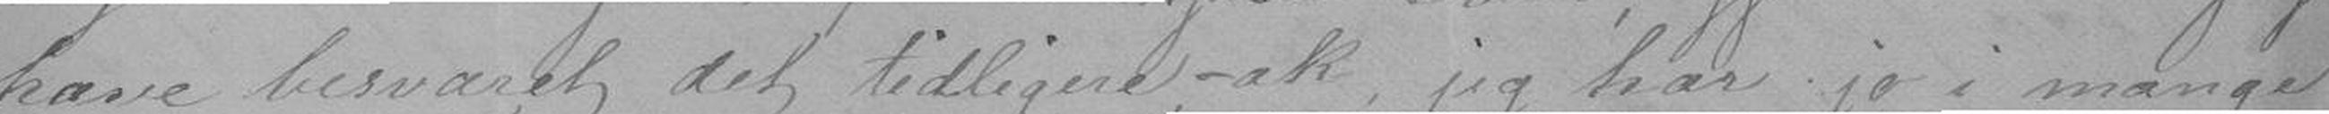have besvaret det tidligere, - ak, jeg har jo i mange
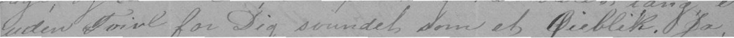uden Tvivl for dig svundet som et Øieblik. Ja
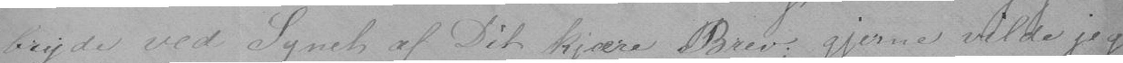bryde ved Synet af Dit kjære Brev; gjerne vilde jeg
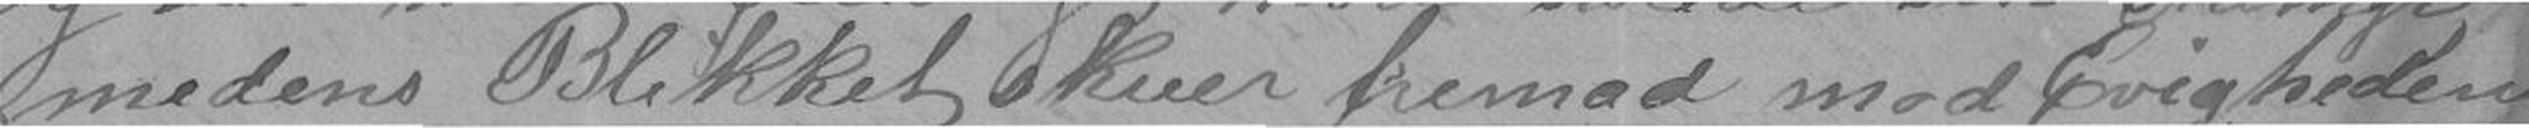medens Blikket skuer fremad mod Evighedens
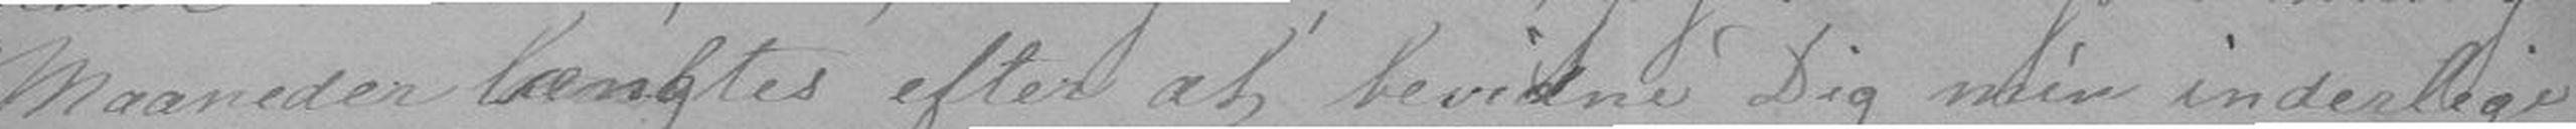Maaneder længtes efter at bevidne Dig min inderlige
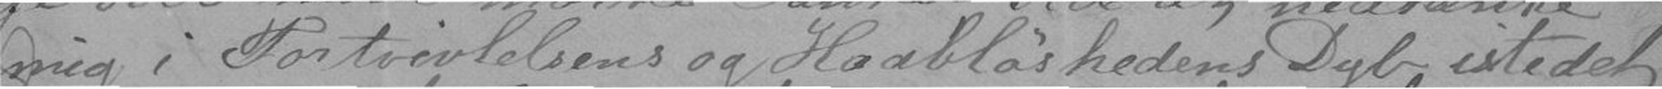mig i Fortvivlelsens og Haabløshedens Dyb istedet
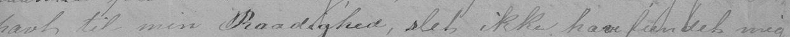havt til min Raadighed, slet ikke have fundet mig
